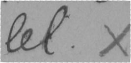lel. x
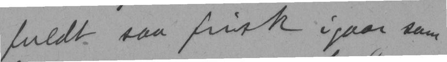fuldt saa frisk igaar som
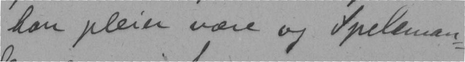han pleier være og Speleman-
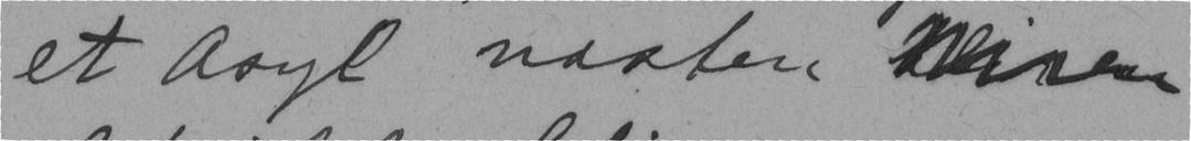et Asyl næsten
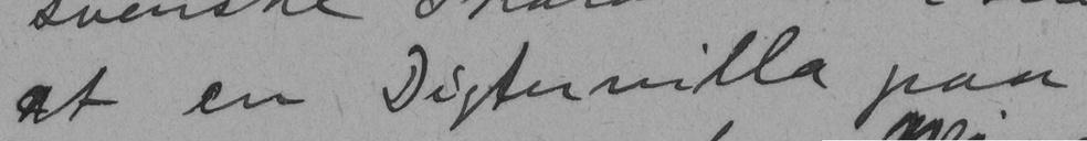at en Digtervilla paa
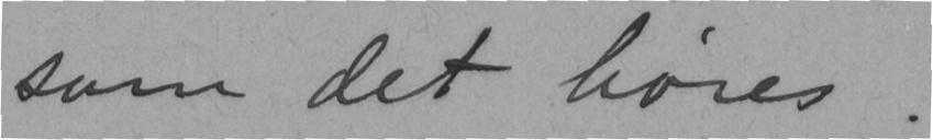som det høres.
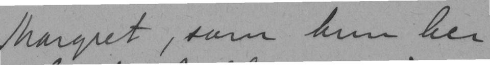Margret, som hun her
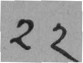22
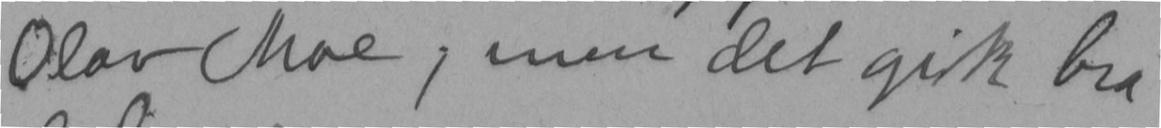Olav Moe; men det gik bra
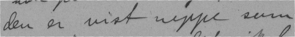den er vist neppe som
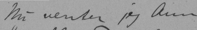Nu venter jeg Ann
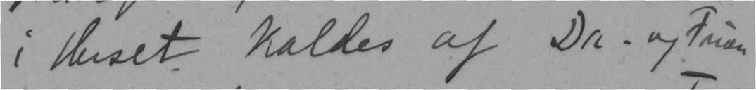i Huset kaldes af Dr. og Fruen
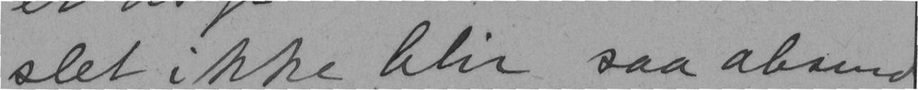slet ikke blir saa absurd
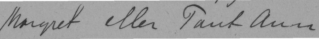Margret eller Tant Ann
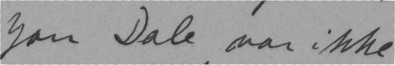Jon Dale var ikke
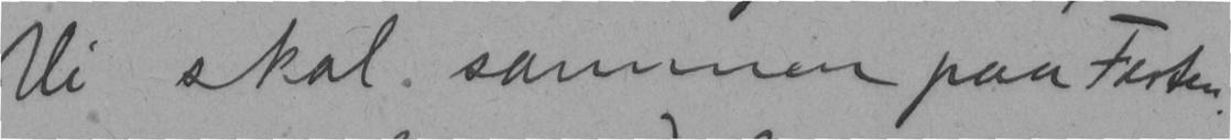Vi skal sammen paa Festen.
